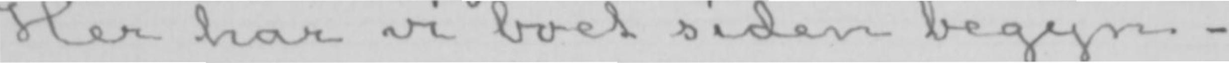Her har vi boet siden begyn-
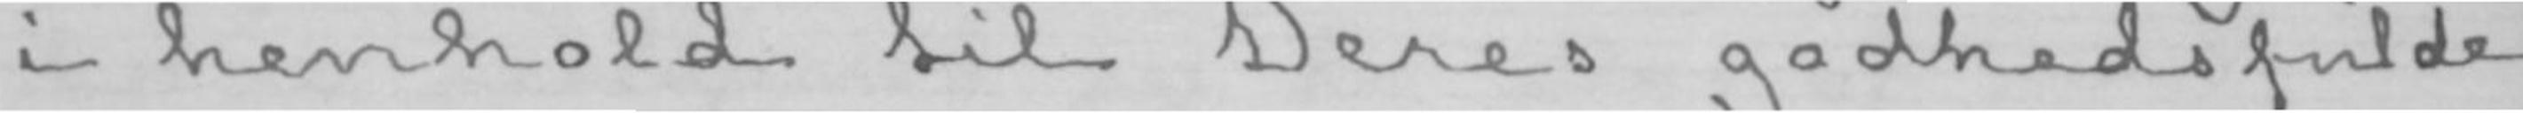i henhold til Deres godhedsfulde
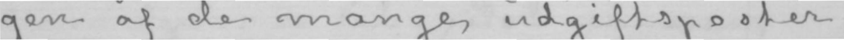gen af de mange udgiftsposter,
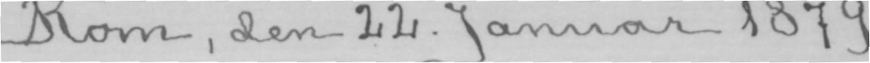Rom, den 22. Januar 1879.
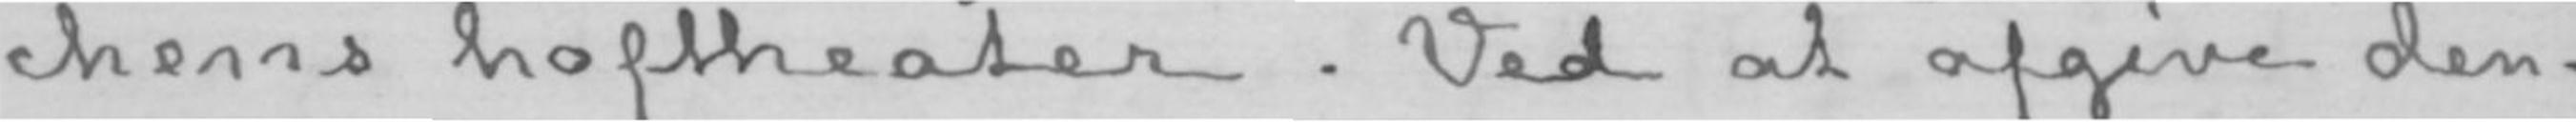chens hoftheater. Ved at afgive den-
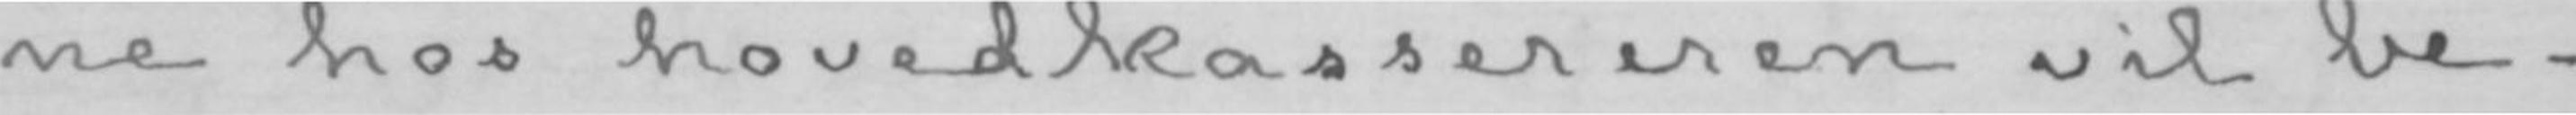ne hos hovedkassereren vil be-
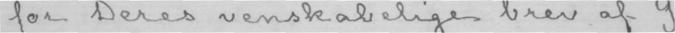for Deres venskabelige brev af 9.
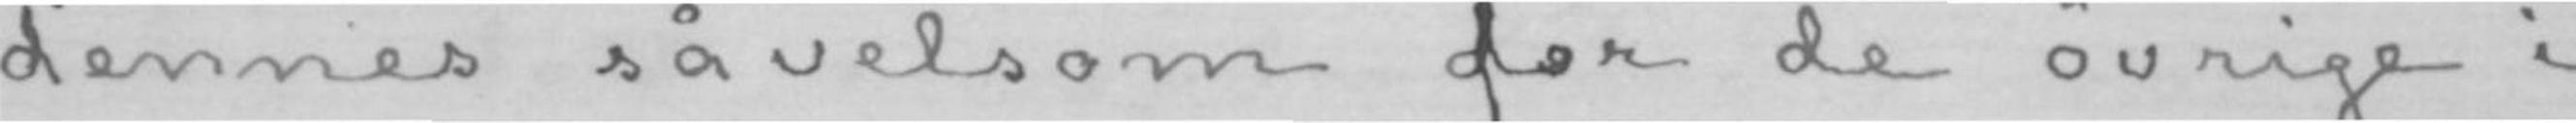dennes såvelsom dfor de øvrige i
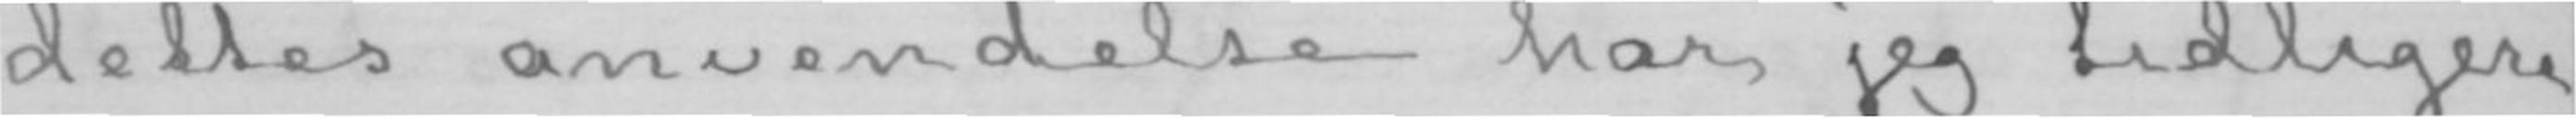dettes anvendelse har jeg tidligere
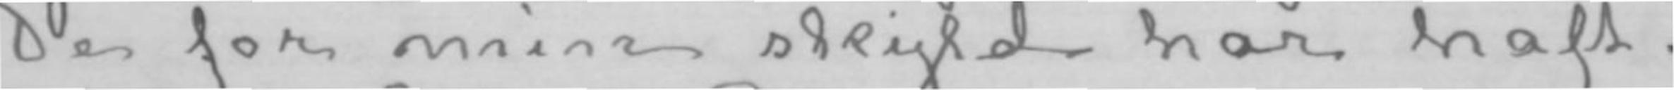De for min skyld har haft.
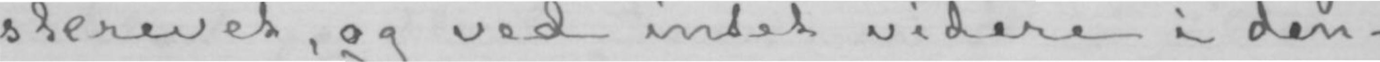skrevet, og ved intet videre i den-
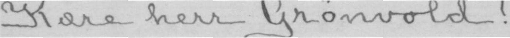Kære herr Grønvold!
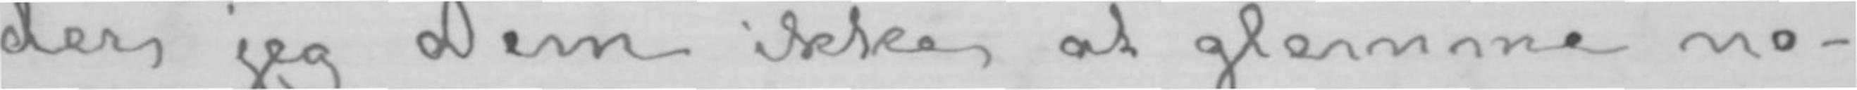der jeg Dem ikke at glemme no-
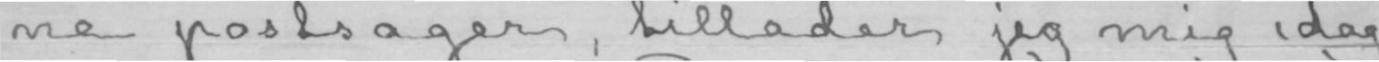ne postsager, tillader jeg mig idag
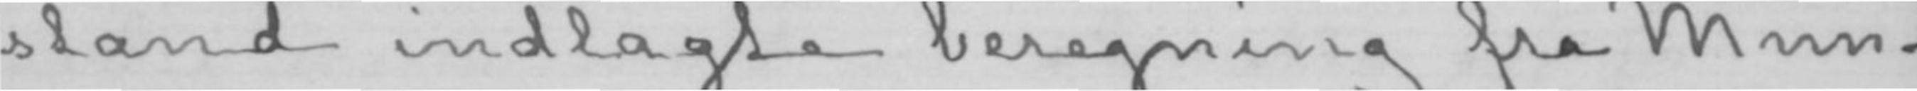stand indlagte beregning fra Mün-
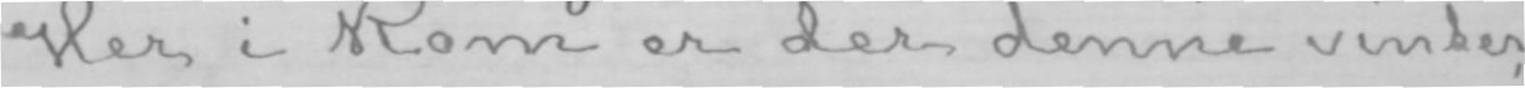Her i Rom er der denne vinter,
